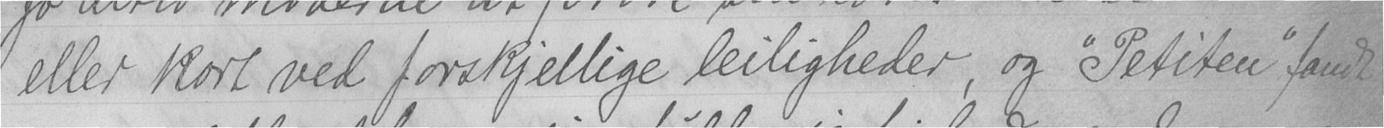eller kort ved forskjellige leiligheder, og "Petiten" fandt
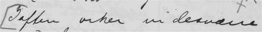Iaften orker vi desværre
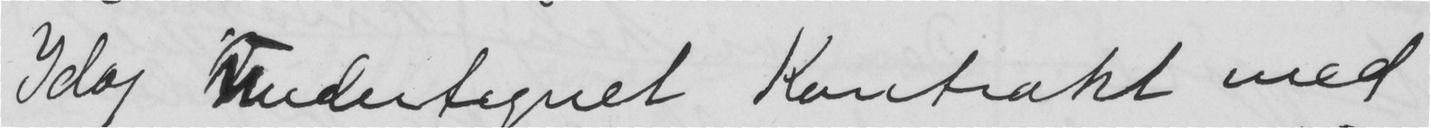Idag Undertegnet Kontrakt med
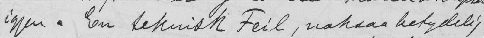igjen. En teknisk Feil, noksaa betydelig
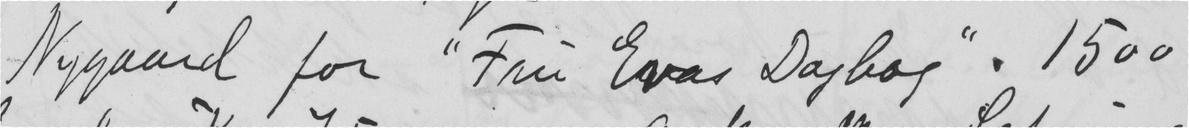Nygaard for "Fru Evas Dagbog". 1500
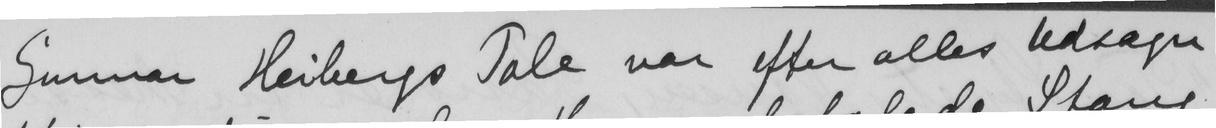Gunnar Heibergs Tale var efter alles Udsagn
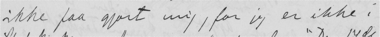ikke faa gjort mig, for jeg er ikke i
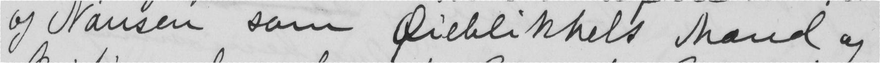og Nansen som Øieblikkets Mand og
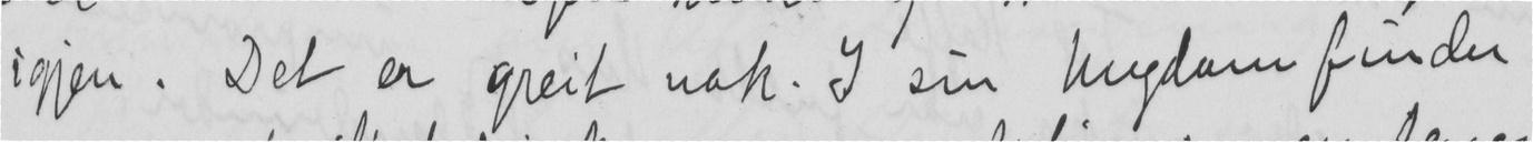igjen. Det er greit nok. I sin Ungdom finder
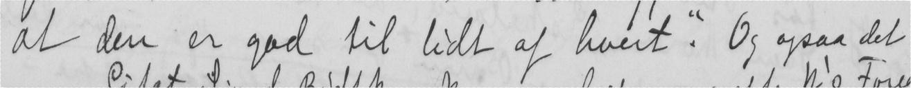at den er god til lidt af hvert." Og ogsaa det
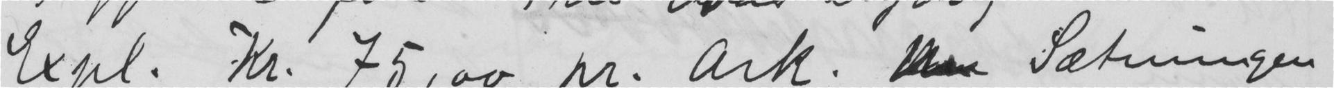Expl. Kr. 75,00 pr. Ark.  Sætningen
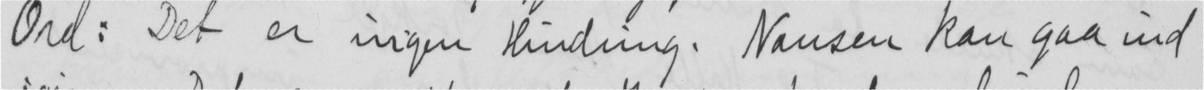Ord: Det er ingen Hindring. Nansen kan gaa ind
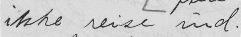ikke reise ind.
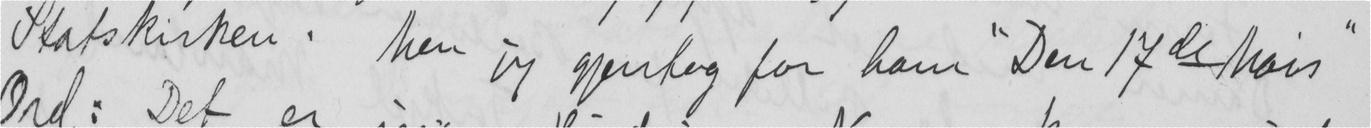Statskirken. Men jeg gjentog for ham "Den 17de Mais"
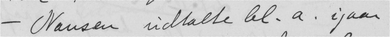- Nansen udtalte bl.a. igaar
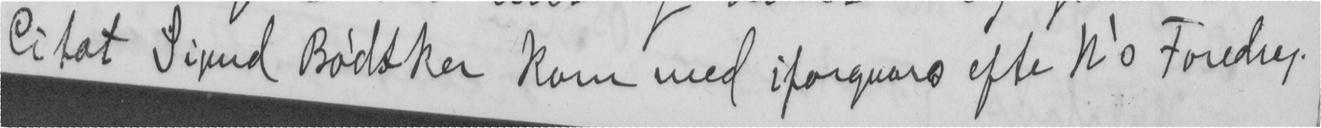Citat Sigrud Bødtker kom med iforgaars efte N's Foredrag.
